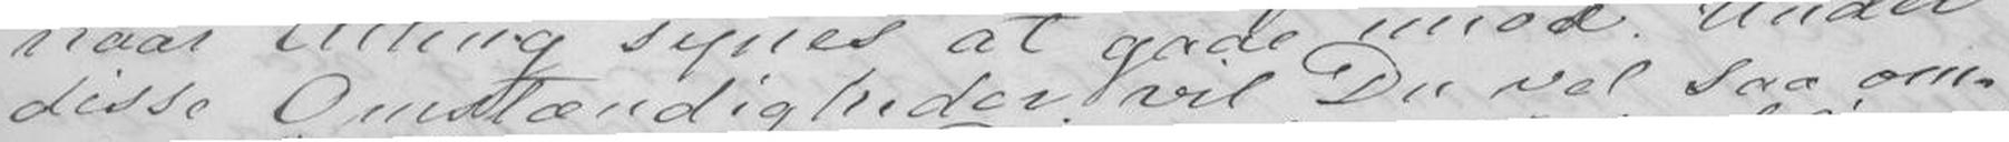disse Omstændigheder vil Du vel saa om.
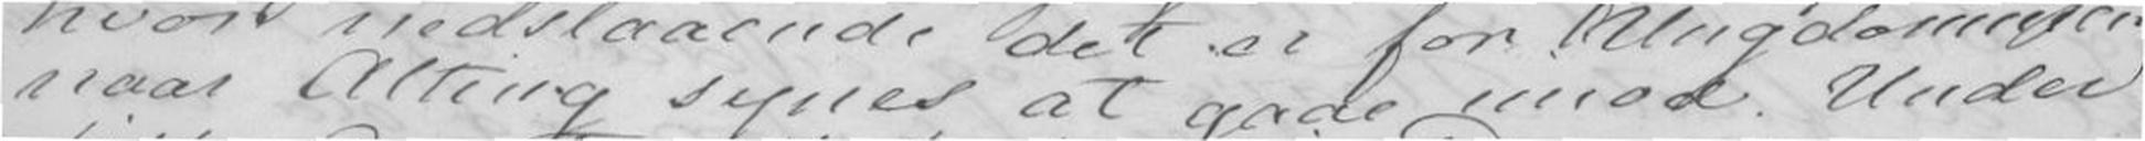naar Alting synes at gaae imod. Under
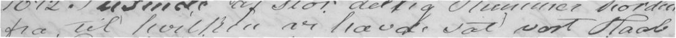fra, til hvilken vi havde sat vort Haab
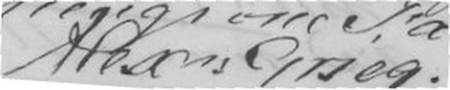Alexr. Grieg.
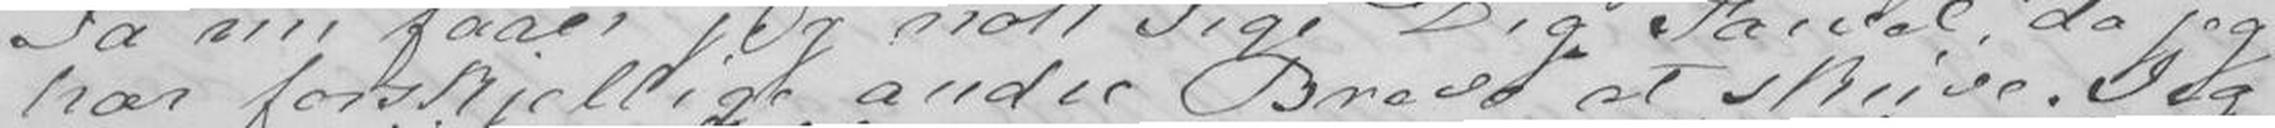har forskjellige andre Breve at skrive. Jeg
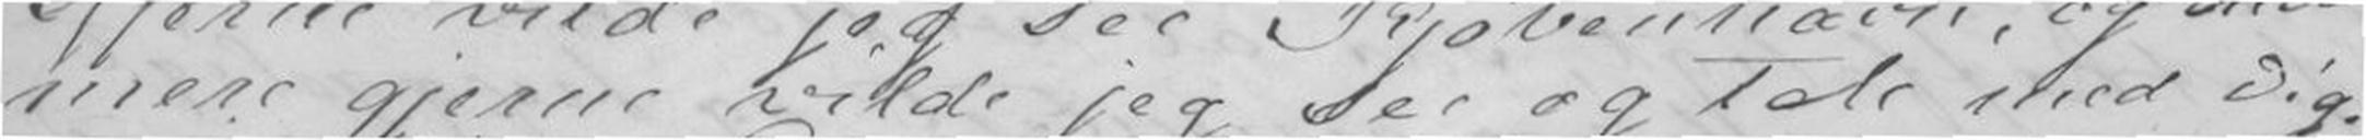mere gjerne vilde jeg see og tale med Dig,
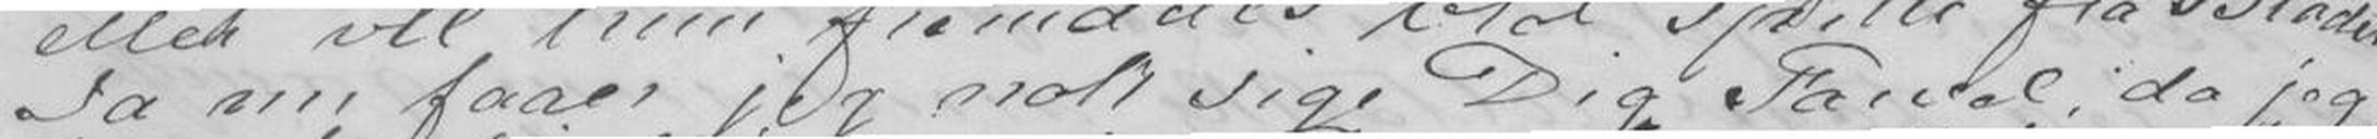Ja nu faaer jeg nok sige Dig Farvel, da jeg
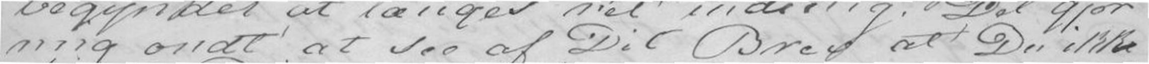mig ondt at see af Dit Brev at Du ikke
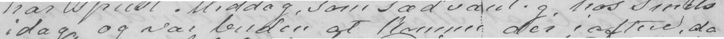idag, og var buden at komme der iaften, da
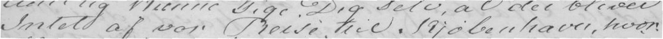Intet af vor Reise til Kjøbenhavn, hvor-
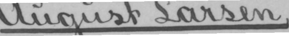August Larsen,
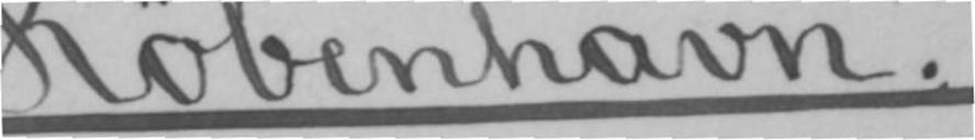København.
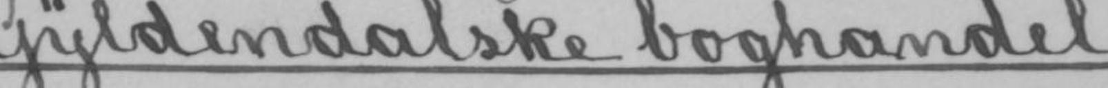Gyldendalske boghandel,
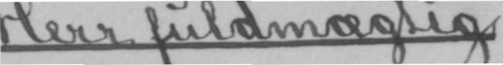Herr fuldmægtig
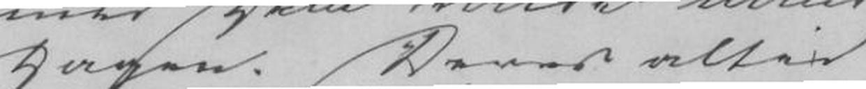Dagen. Deres altid
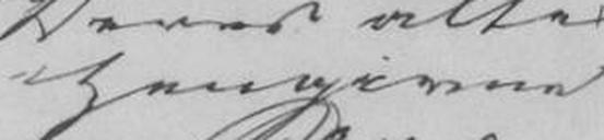hengivne
